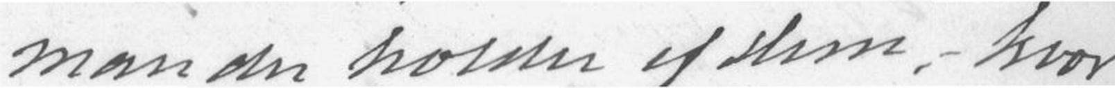man da holder af Dem, - hvor
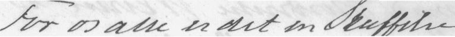For os alle er det en Skuffelse
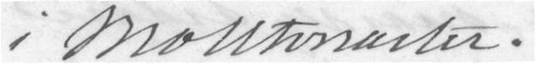i Molltonarter.
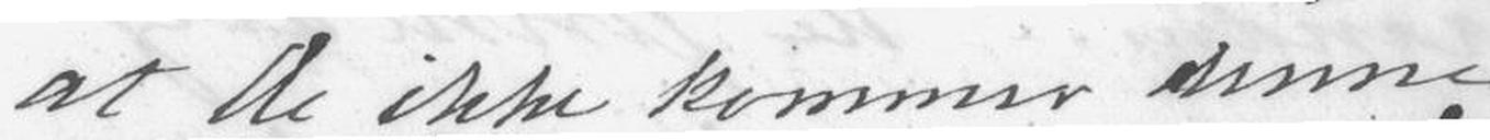at De ikke kommer denne
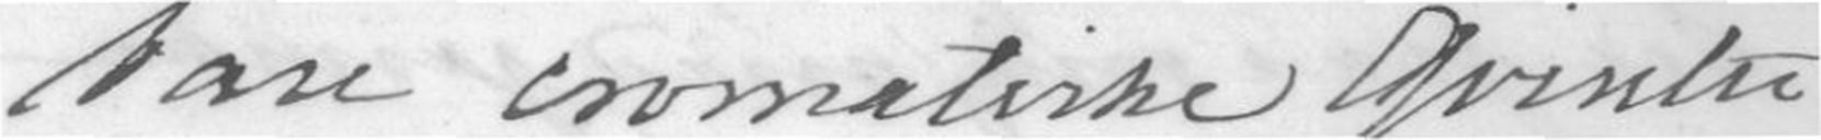bare cromatiske Qvintu
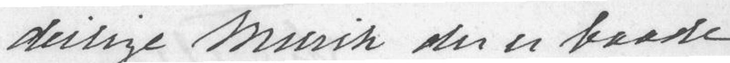deilige Musik der er baade
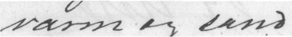varm og sand
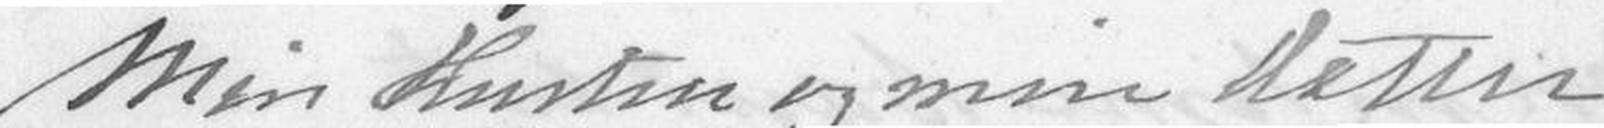Min Hustru og min Datter
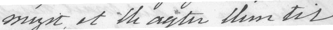meget. at thi agter Dem til
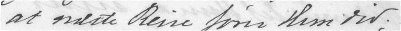at næste Reise fører Dem did;
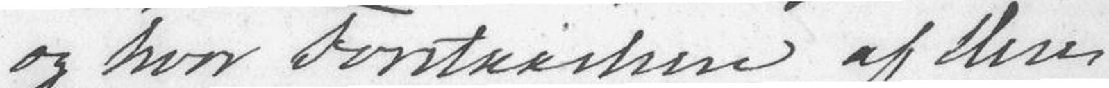og hvor Forstaashen af Deres
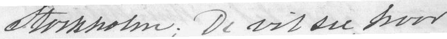Stockholm; De vil see, hvor
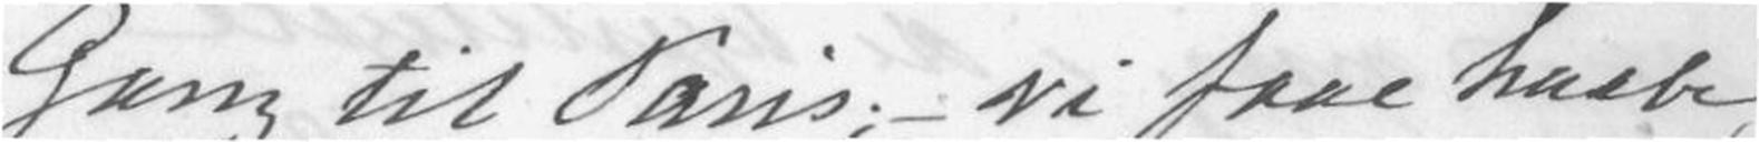Gang til Paris; - vi faar haabe,
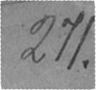271.
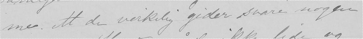me. At de virkelig gider svare nogen
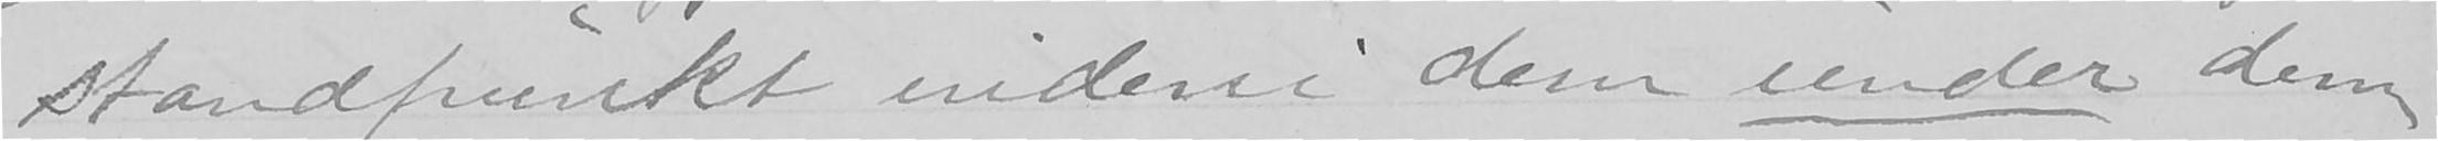standpunkt indeni dem under dem
